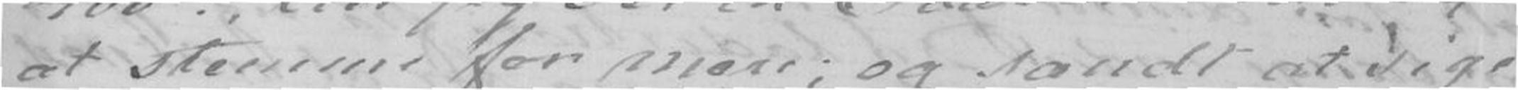at stemme for mere, og sandt at sige
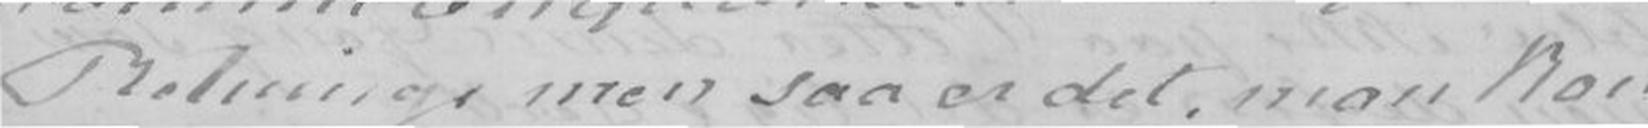Retning, men saa er det, man kan
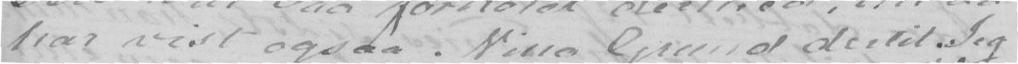har vist ogsaa Nina Grund der til. Jeg
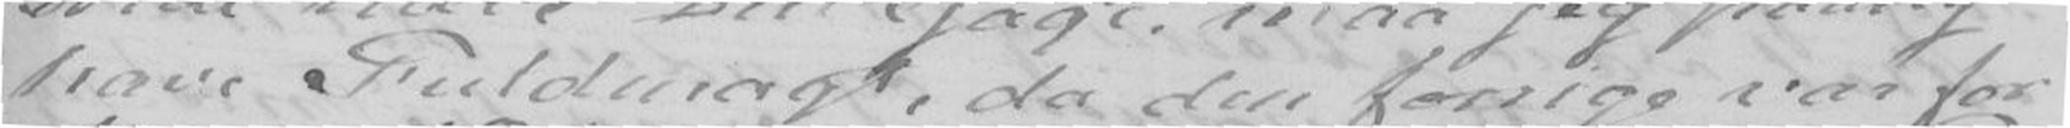have Fuldmagt, da den forrige var for
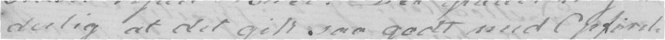derlig at det gik saa godt med Opførel-
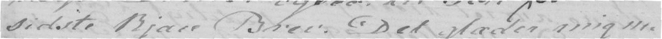sidste kjære Brev. Det glæder mig in-
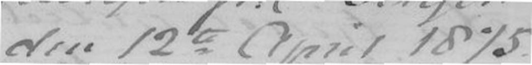den 12te April 1875.
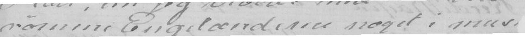rømme Englænderne noget i mus
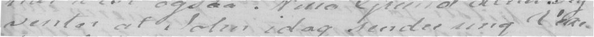venter at John idag sender mig Vexe-
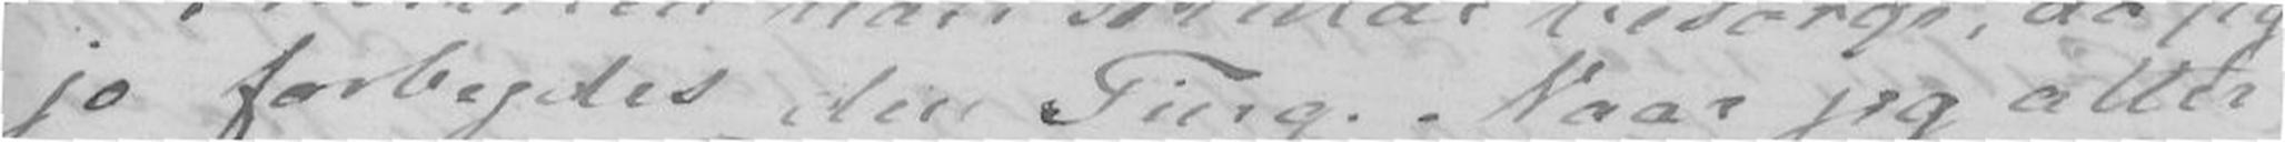jo forbydes den Ting. Naar jeg atter
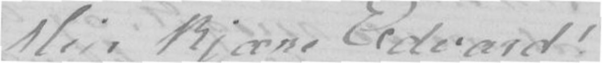Min kjære Edvard!
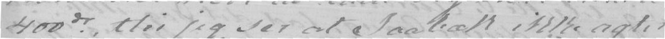400do, thi jeg ser at Jaabæk ikke agter
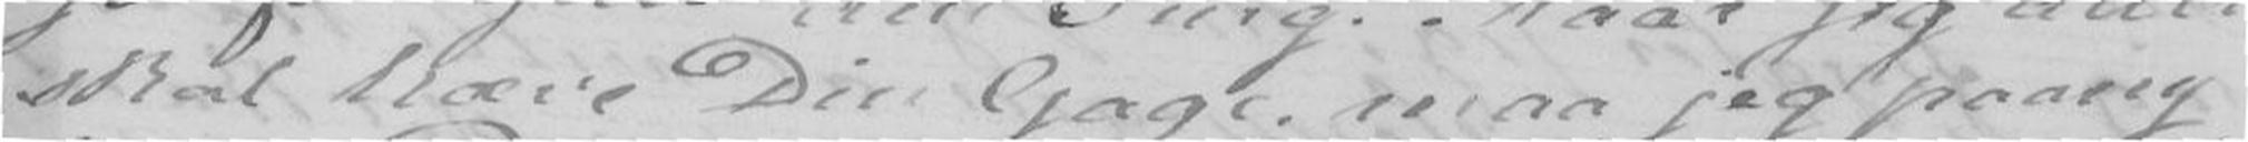skal hæve Din Gage, maa jeg paany
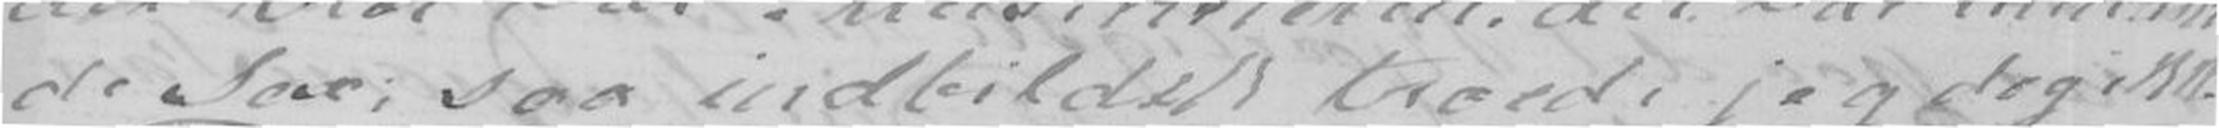de Sex; saa indbildsk troede jeg dog ikke
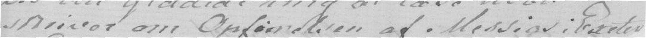skriver om Opførelsen af Messias i Exct
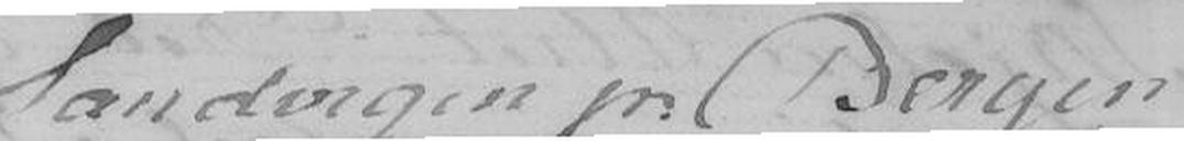Sandvigen pr Bergen
